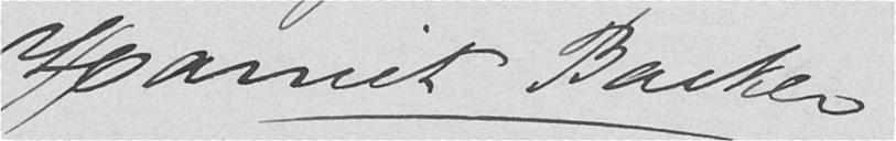Harriet Backer
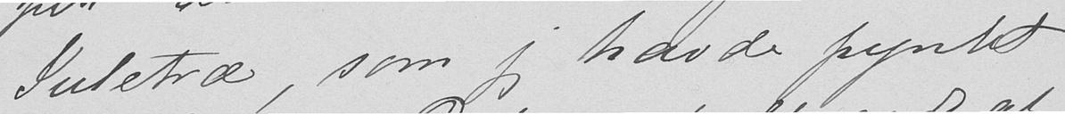Juletræ, som jeg havde pyntet
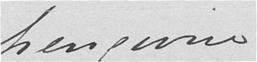hengivne
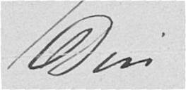Din
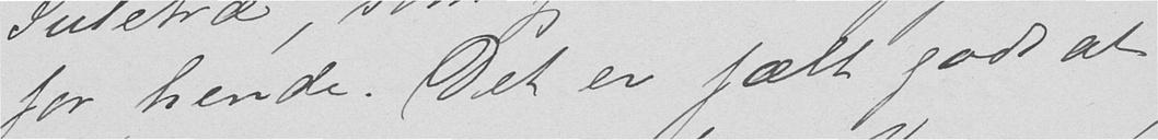for hende. Det er fælt godt at
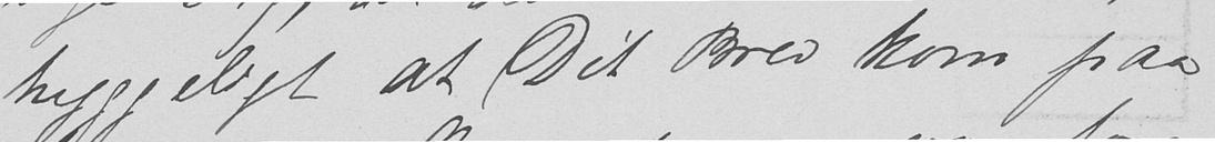hyggeligt at Dit Brev kom paa
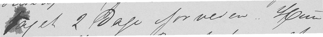kaget 2 Dage forveien. Hun
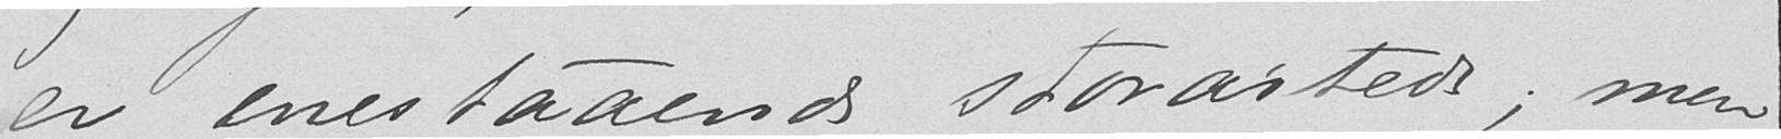er enestaaende storartede; men
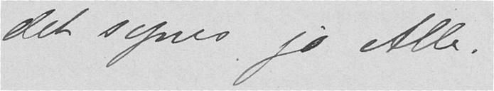det synes jo Alle.
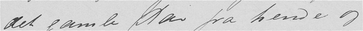det gamle Aar fra hende og
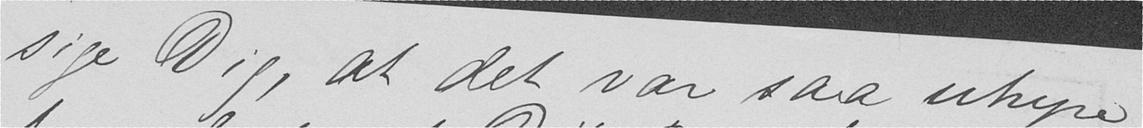sige Dig, at det var saa uhyre
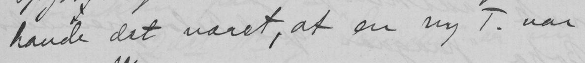havde det været, at en ny T. var
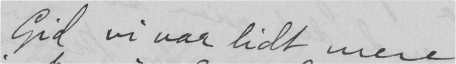Gid vi var lidt mere
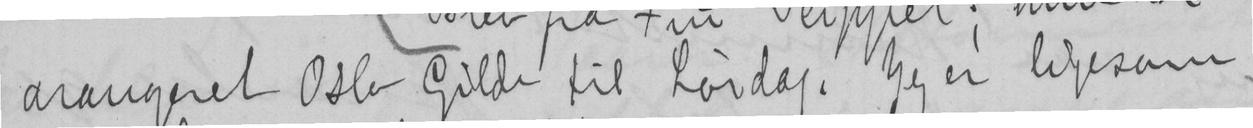arangeret Oslo Gilde til Lørdag. Jeg er ligesom
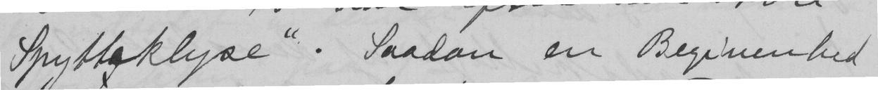Spytteklyse". Saadan en Begivenhed
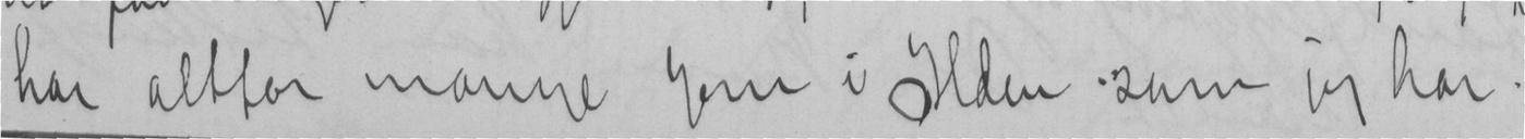har altfor mange jern i ilden som jeg har.
